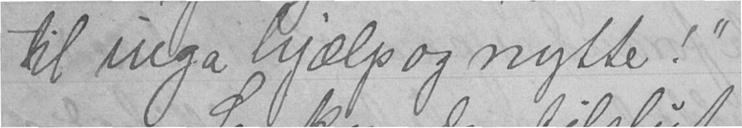til inga hjælp og nytte!"
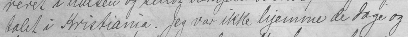talet i Kristiania. Jeg var ikke hjemme de dage og
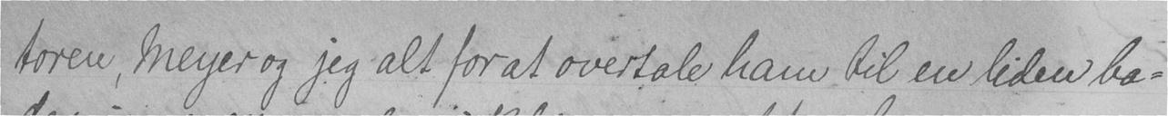toren, Meyer og jeg alt for at overtale ham til en liden ba-
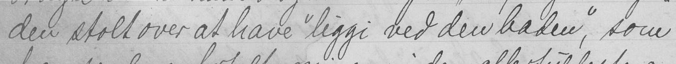den stolt over at have "liggi ved den baden," som
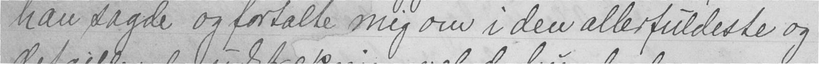han sagde og fortalte mig om i den allerfuldeste og
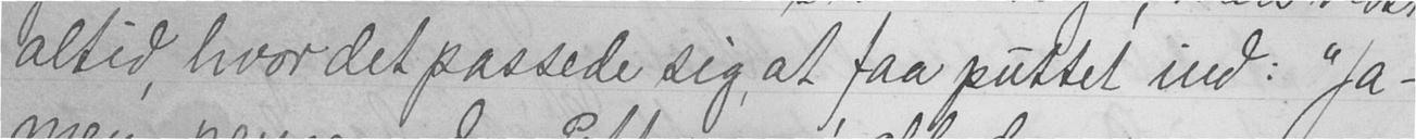altid, hvor det passede sig, at faa puttet ind: "Ja-
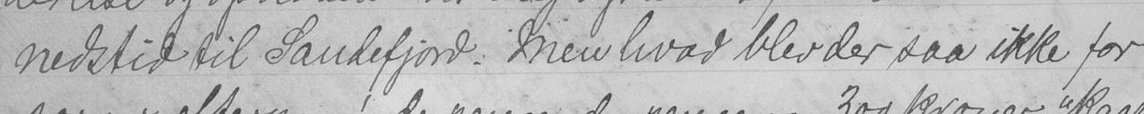nedstid til Sandefjord. Men hvad blev der saa ikke for
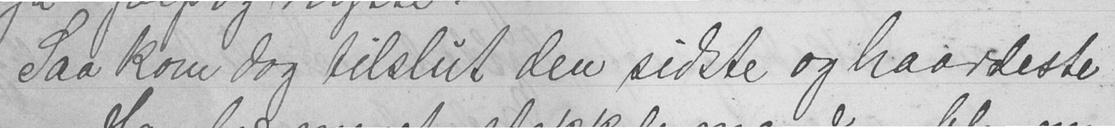Saa kom dog tilslut den sidste og haardeste
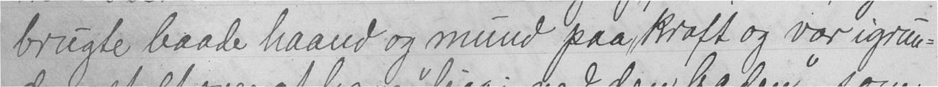brugte baade haand og mund paa kraft og var igrun-
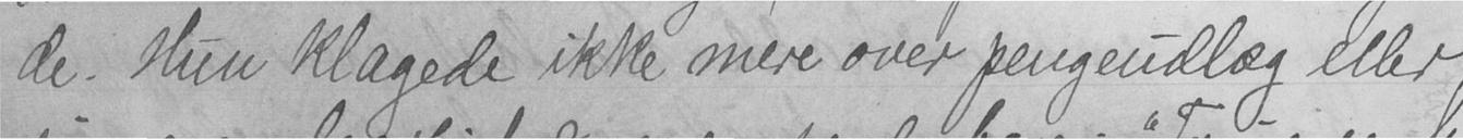de. Hun klagede ikke mere over pengeudlæg eller
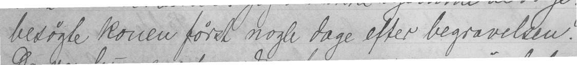besøgte konen først nogle dage efter begravelsen.
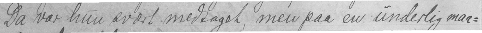Da var hun svært medtaget, men paa en underlig maa-
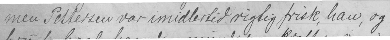men Pettersen var imidlertid rigtig frisk, han, og
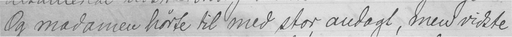Og madamen hørte til med stor andagt, men vidste
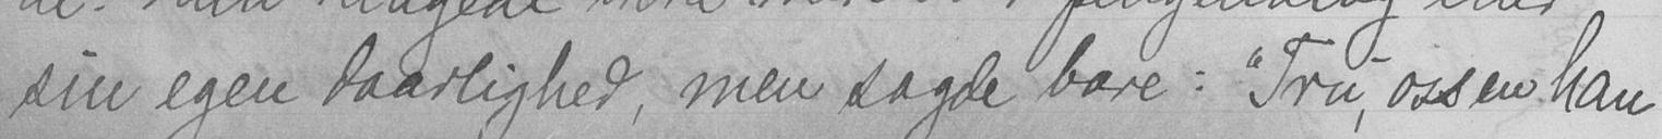sin egen daarlighed, men sagde bare: "Tru, ossen han
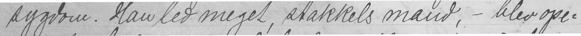sygdom. Han led meget, stakkels mand, - blev ope-
